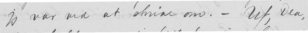jeg var ved at skrive om. - Uf, Dea,
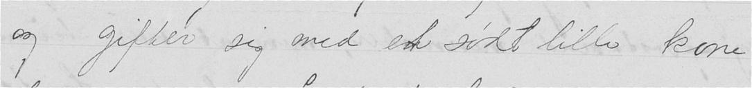og gifter sig med et sødt lille kone-
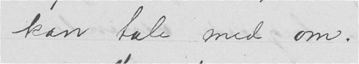kan tale med om.
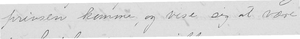prinsen komme, og vise sig at være
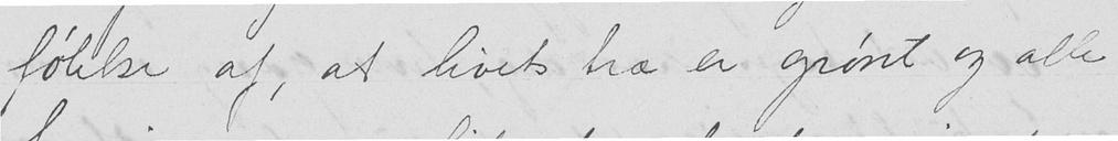følelse af, at livets træ er grønt og alle
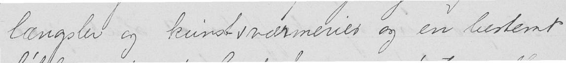længsler og kunstsværmerier og en bestemt
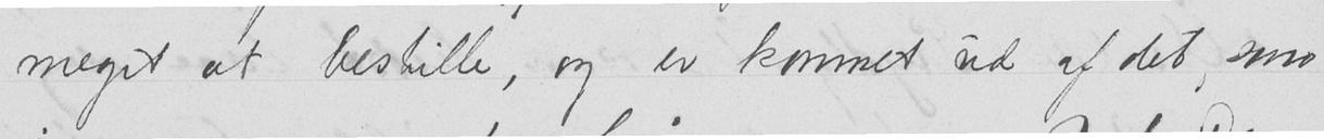meget at bestille, og er kommet ud af det som
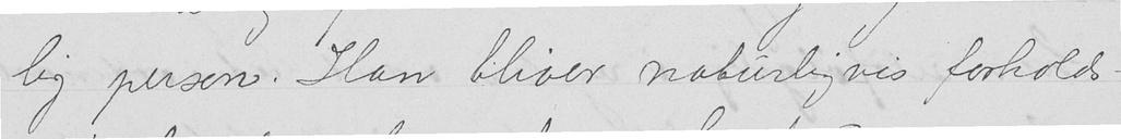lig person. Han bliver naturligvis forholds-
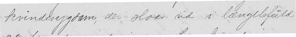kvindesygdom, der slaar ud i længselsfuld
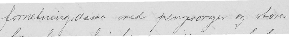forretningsdame med pengesorger og store
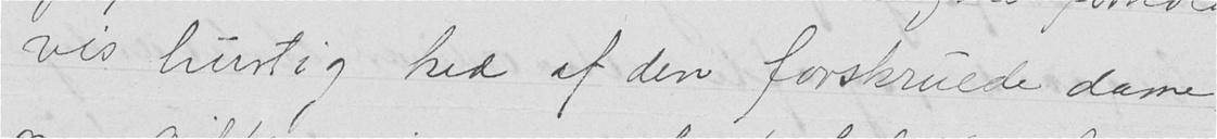vis hurtig ked af den forskruede dame
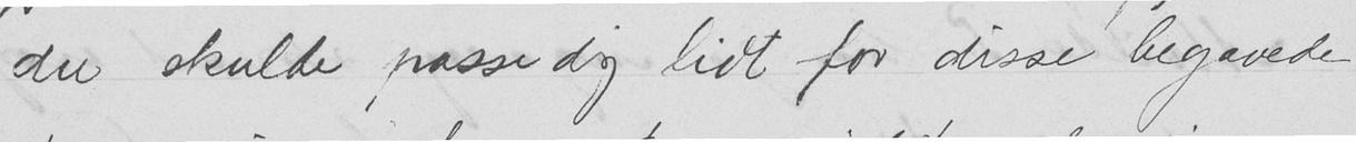du skulde passe dig lidt for disse begavede
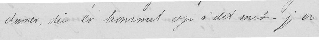damer, du er kommet op i det med - jeg er
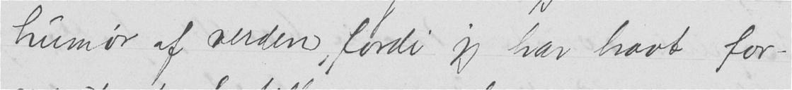humør af verden, fordi jeg har havt for-
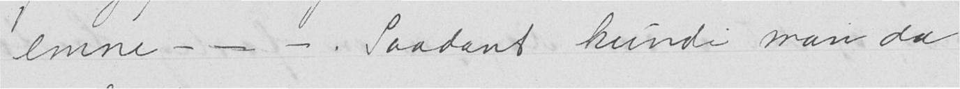emne - - -. Saadant kunde man da
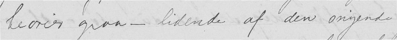teorier graa - lidende af den snigende
